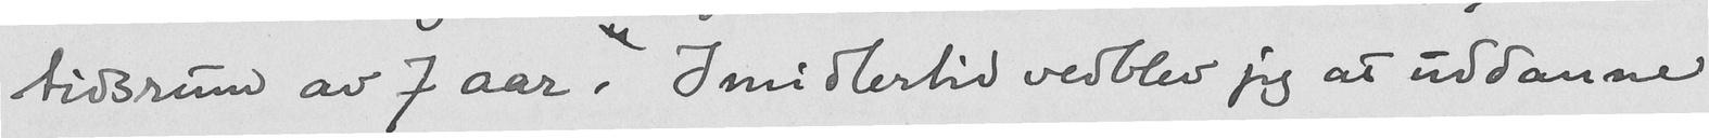tidsrund av 7 aar. Imidlertid vedblev jeg at uddanne
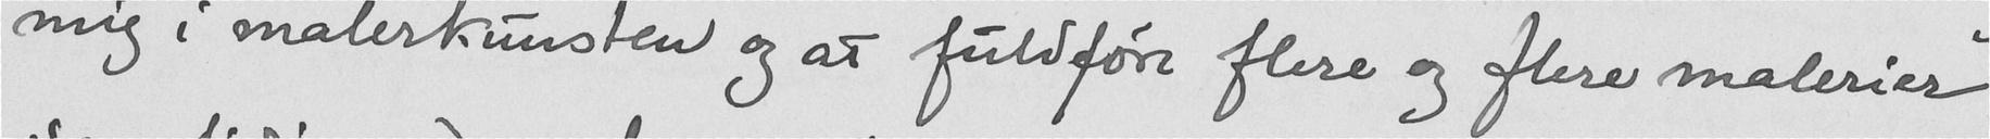mig i malerkunsten og at fuldføre flere og flere malerier"
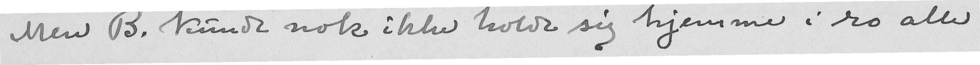Men B. kunde nok ikke holde sig hjemme i ro alle
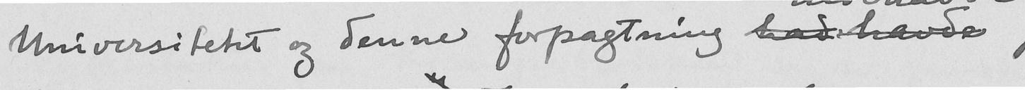Universitetet og denne forpagtning had havde
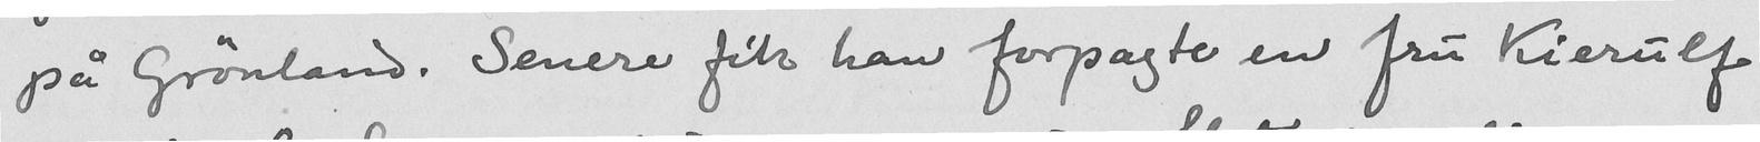på Grønland. Senere fik han forpagte en fru Kierulf
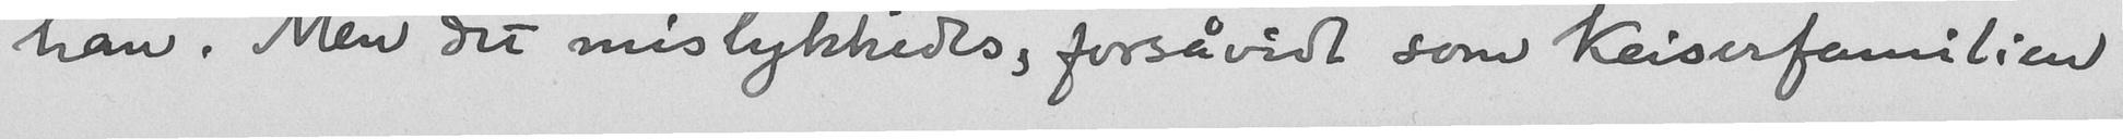han. Men det mislykkedes, forsåvidt som keiserfamilien
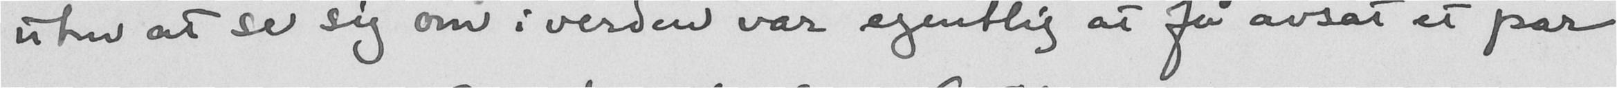uten at se sig om i verden var egentlig at få avsat et par
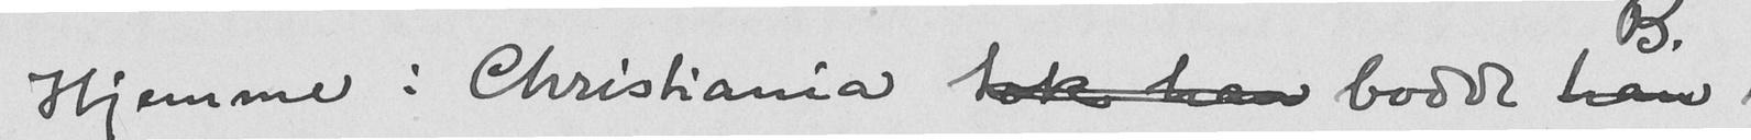Hjemme i Christiania tok han bodde han
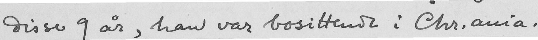disse 9 år, han var bosittende i Chr.ania.
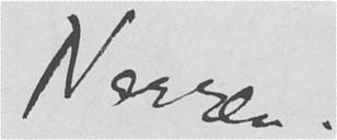Narren.
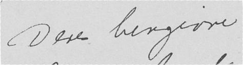Deres hengivne
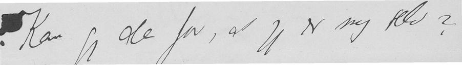Kan jeg da for, at jeg er meg selv?
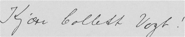Kjære Collet Vogt!
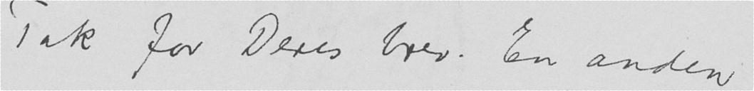Tak for Deres brev. En anden
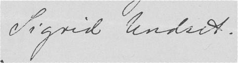Sigrid Undset.
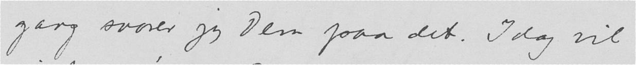gang svarer jeg Dem paa det. Idag vil
